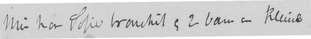Nu har Sofie bronchit og 2 barn er kleine,
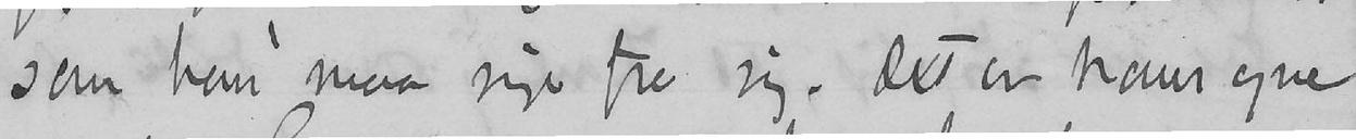som han maa sige fra sig. Det er hans egne
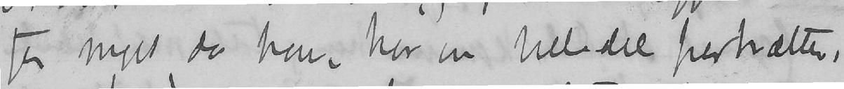for meget, da han har en hel del portrætter,
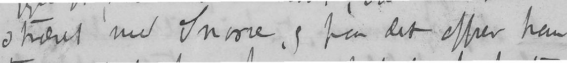strævet med Snorre, og paa det offrer han
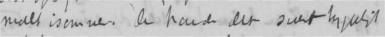malt isommer. De havde det svært hyggeligt
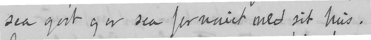saa godt og er saa fornøiet med sit hus.
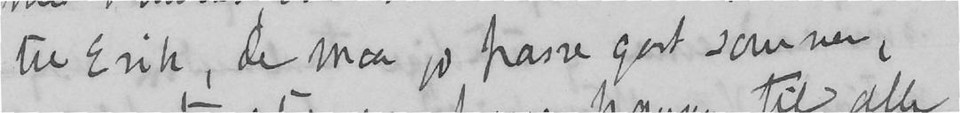til Erik, de maa jo passe godt sammen,
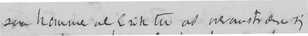saa kommer vel Erik til at overanstrænge sig
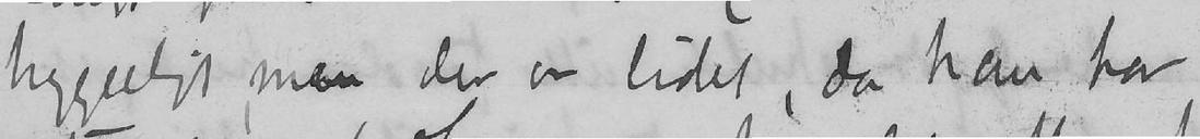hyggeligt, men der er lidet, da han har
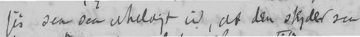før saa saa uheldigt ud, at den skyder saa
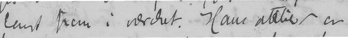langt frem i værket. Hans atelier er
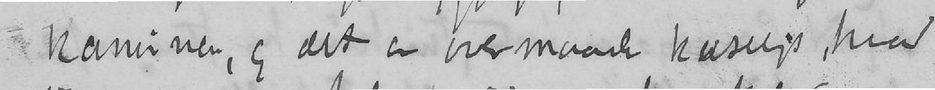kaminen, og det er overmaade kuseligt, hvad
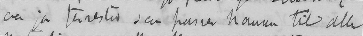aa ja forresten saa passer Nansen til alle
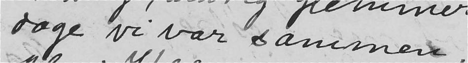dage vi var sammen.
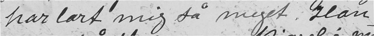har lært mig så meget. Han
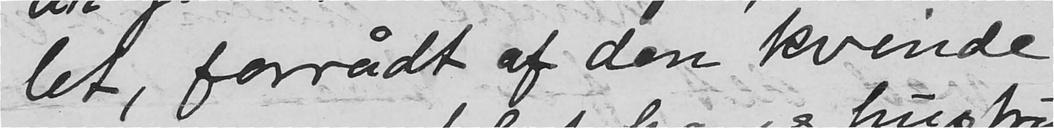let, forrådt af den kvinde
